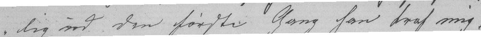lig ud den første Gang han traf mig.
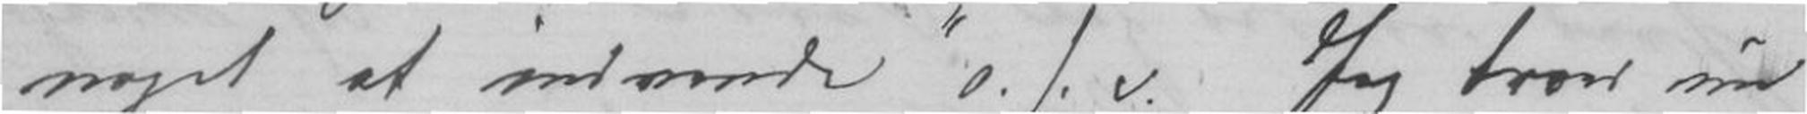noget at indvende o. s. v. Jeg tror nu
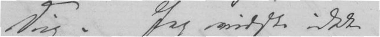Dig. Jeg vidste ikke
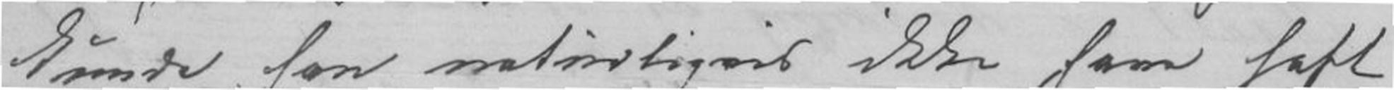kunde han naturligvis ikke have haft
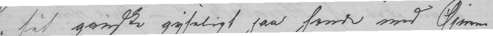sit ganske gyseligt paa hende med Øjnene,
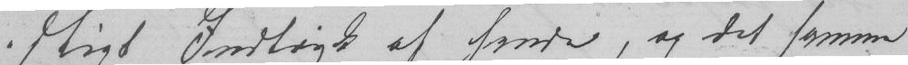stigt Indtryk af hende, og det samme
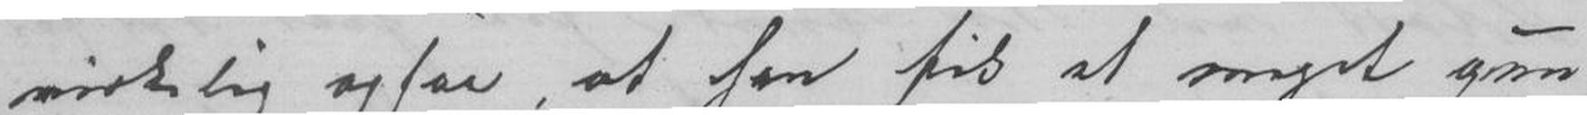virkelig ogsaa, at han fik et meget gun-
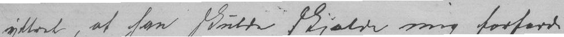at han skulde skjælde mig forfærde-
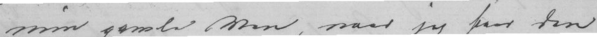min gamle ven, naar jeg faar den
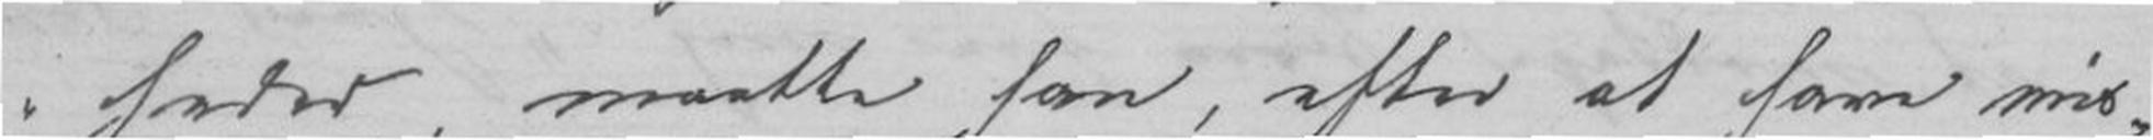heder, maatte han, efter at have -
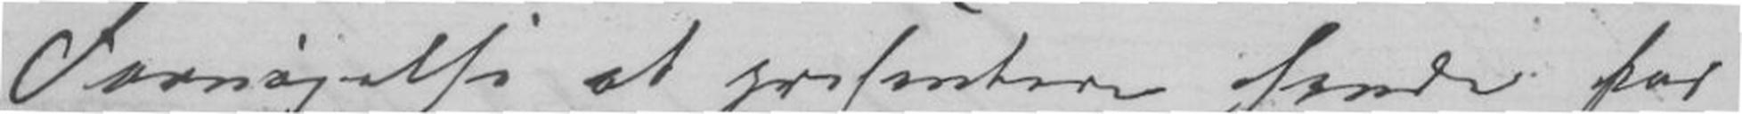Fornøielse at presentere hende for
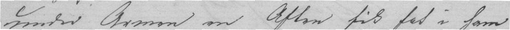under armen om Aften fik fat i ham
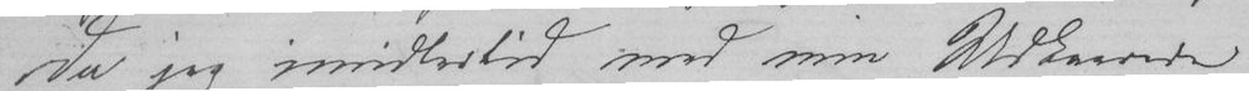Da jeg imidlertid med min Tilkommende
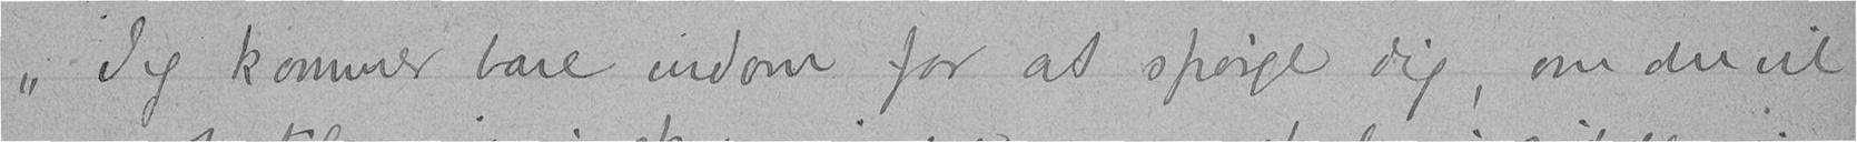"Jeg kommer bare indom for at spørge dig, om du vil
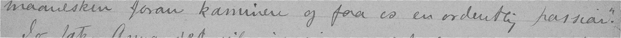maaneskin foran kaminen og faa os en ordentlig passiar".
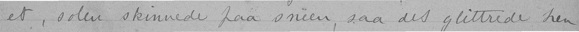et, solen skinnede paa sneen, saa det glittrede hen
